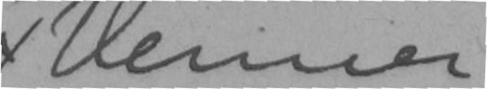venner
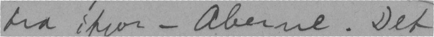fra ifjor - Aberne. Det
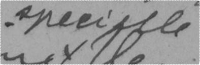specielle
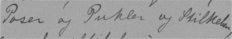Poser og Pukler og Stilkeben,
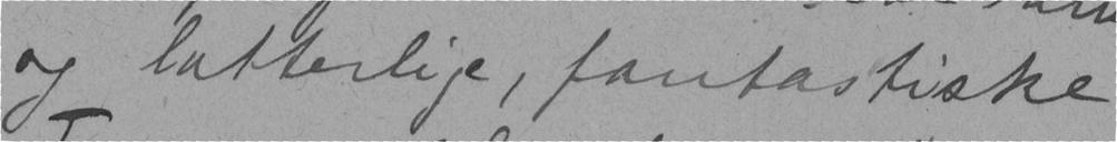og latterlige, fantastiske
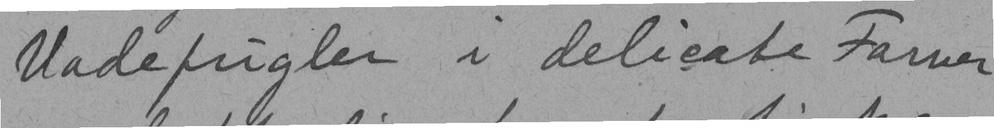Vadefugler i deligate Farver
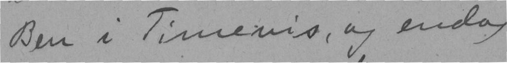Ben i Timevis, og endog
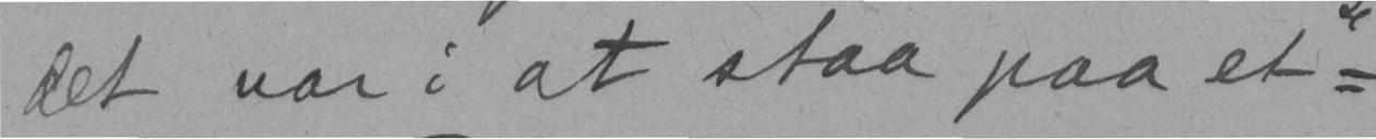det var i at staa paa et -
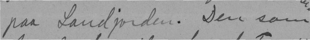paa Landjorden. Den som
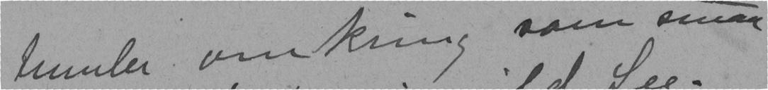tumler omkring som smaa
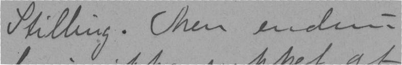Stilling. Men endnu
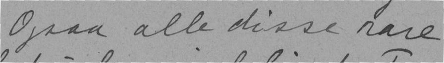Ogsaa alle disse rare
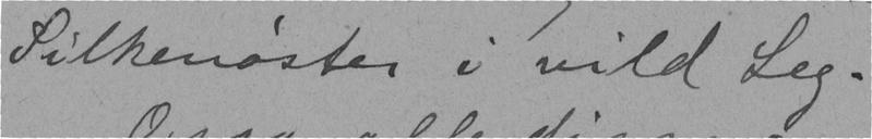Silkenøster i vild Leg.
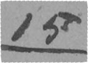15
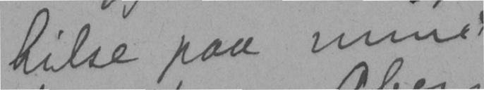hilse paa mine
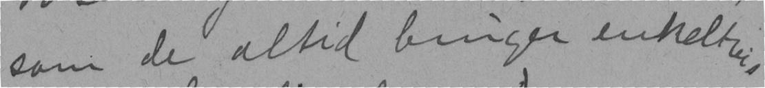som de altid bruger enkeltvis
